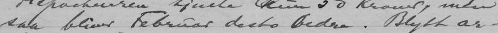saa bliver Februar desto bedre. Blytt ar-
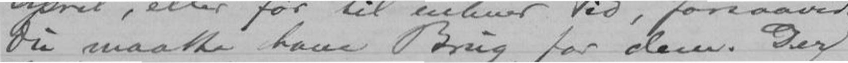Du maatte have Brug for dem. Der
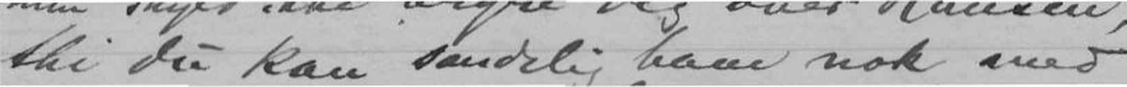thi Du kan sandelig have nok med
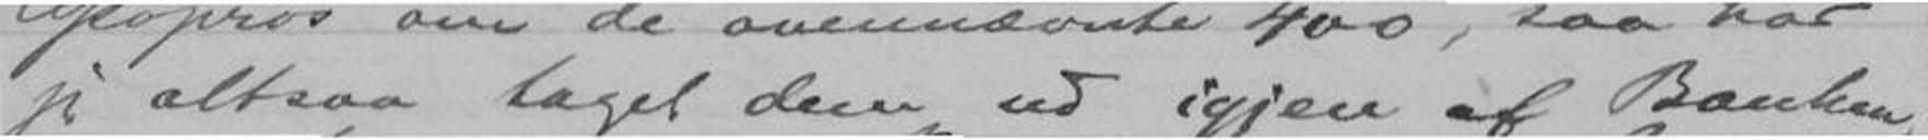jeg altsaa taget dem ud igjen af Banken,
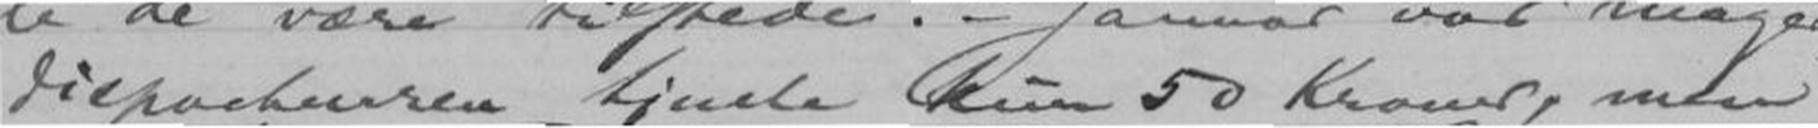Dispacheuren tjente kun 50 kroner, men
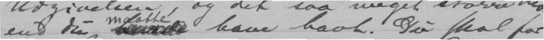end Du maatte have havt. Du skal for
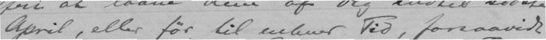April, eller før til enhver Tid, forsaavidt
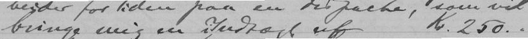bringe mig en Indtægt afKr. 250.-
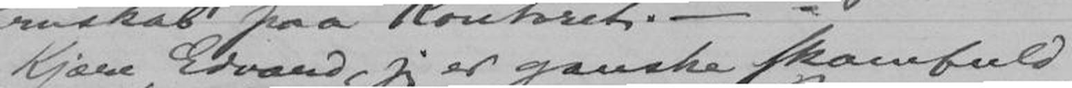Kjære Edvard, jeg er ganske skamfuld
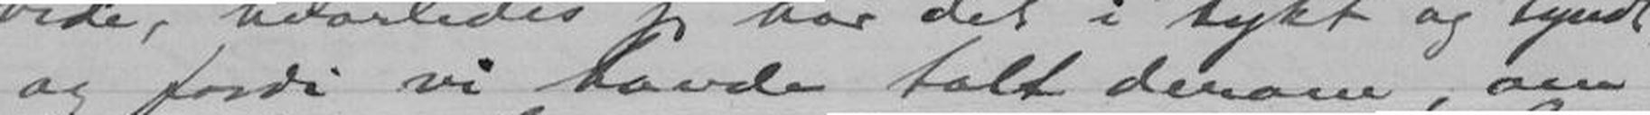og fordi vi havde talt derom, om
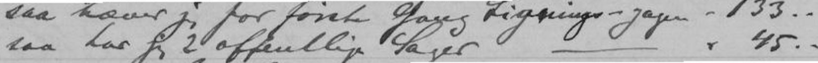saa har jeg 2 offentlige Sager ---------------- " 45.-
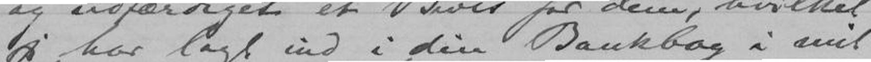jeg har lagt ind i Din Bankbog i mit
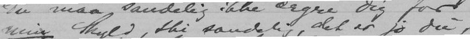min Skyld, thi sandelig, det er jo Du,
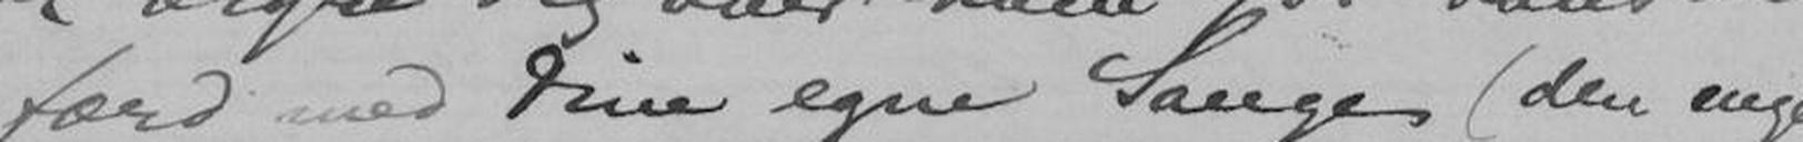færd med Dine egne Sange (den engelske
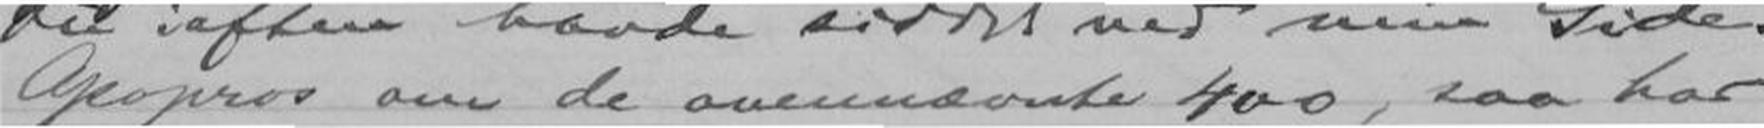Apropos om de ovennævnte 400, saa har
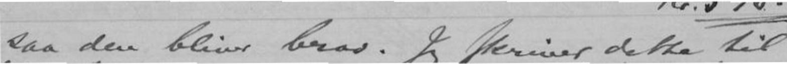saa den bliver brad. Jeg skriver dette til
